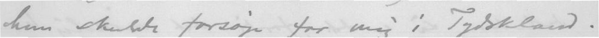hun skulde forsøge for mig i Tydskland.
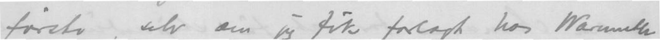første, selv om jeg fik forlagt hos Warmuth
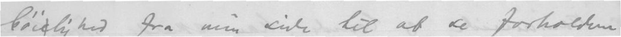bøilighed fra min side til at se forholderne
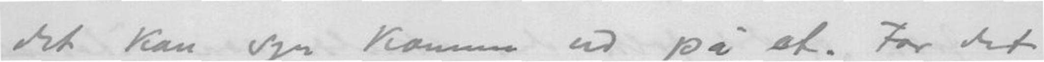det kan syns komme ud på et. For det
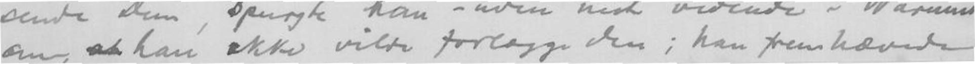om at han ikke vilde forlægge dem; han fremhævede
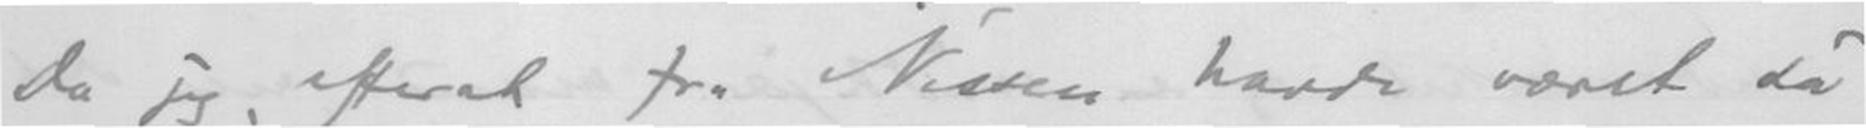Da jeg efterat fr. Nissen havde været så
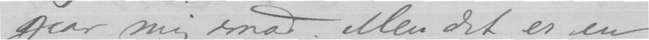gaar mig imod. Men det er en
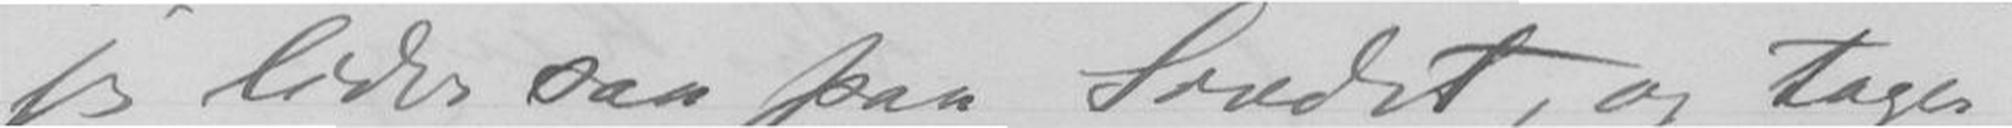jeg lides saa paa Sindet, og tager
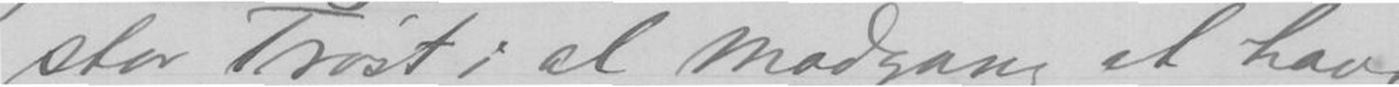stor Trøst i al Modgang at have
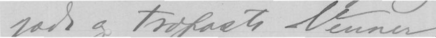gode og trofaste Venner.
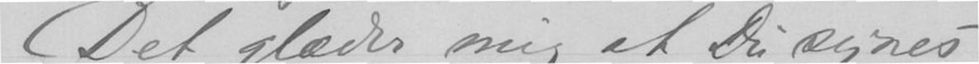Det glæder mig at Du synes
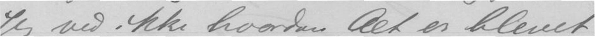Jeg ved ikke hvordan Alt er blevet,
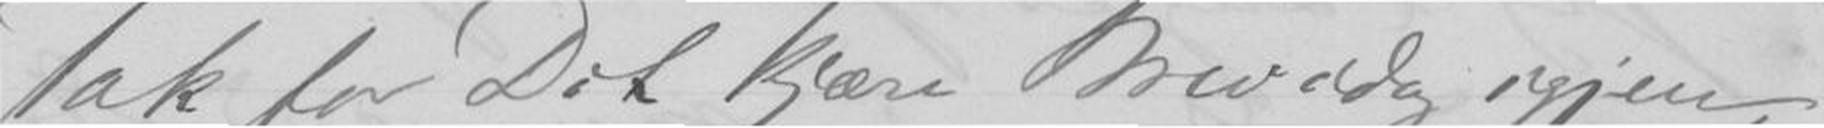Tak for Det kjære Brev idag igjen,
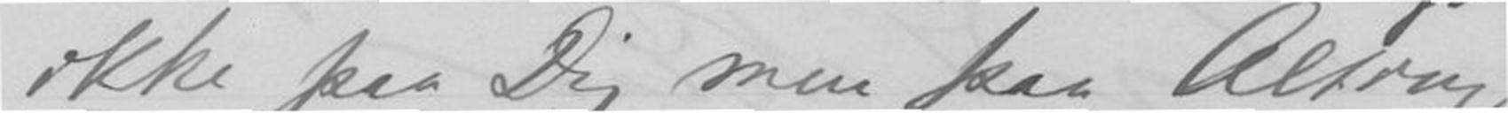ikke paa Dig men paa alting
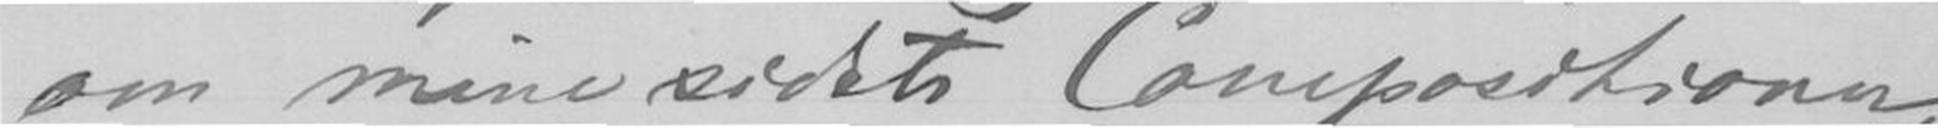om mine sidste Compositioner,
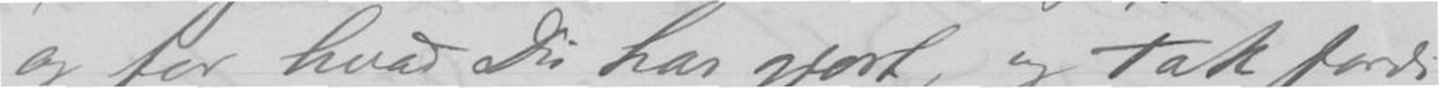og for hvad Du har gjort, og tak fordi
